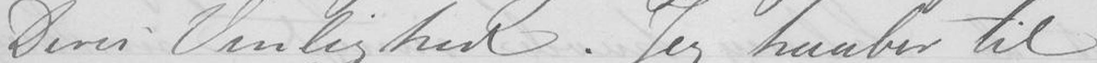Deres Venlighed. Jeg haaber til
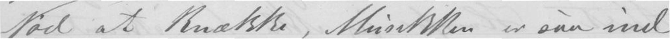Nød at knække, Musikken er saa ind
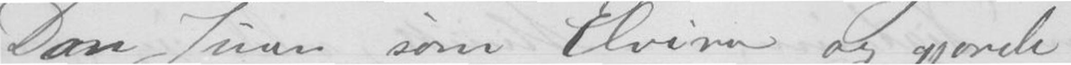i Don Juan som Elvira og gjorde
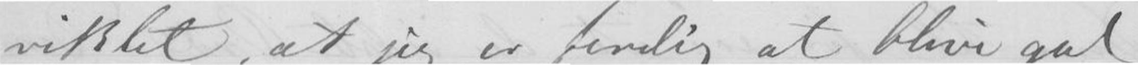viklet at jeg er ferdig at blive gal
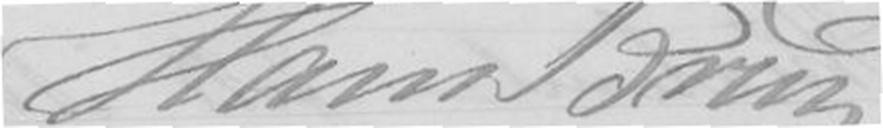Hans Brun
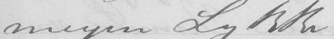megen Lykke.
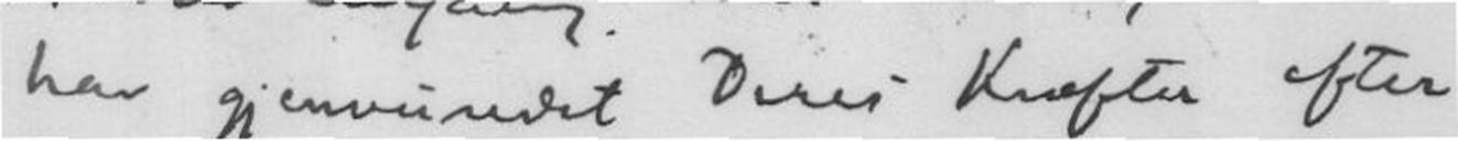har gjenvundet Deres Kræfter efter
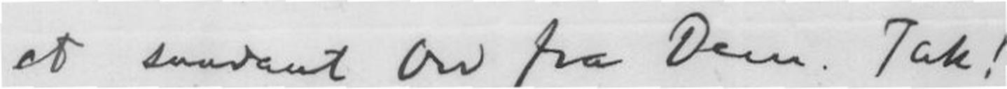et saadant Ord fra Dem. Tak!
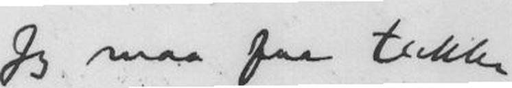Jeg maa faa takke
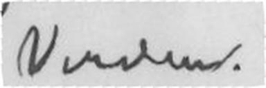Verden.
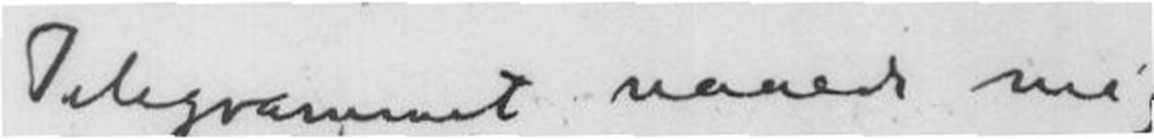Telegrammet naaede mig
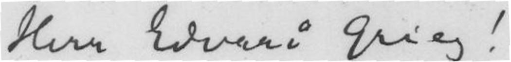Herr Edvard Grieg!
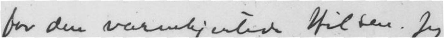for den varmhjertede Hilsen. Jeg
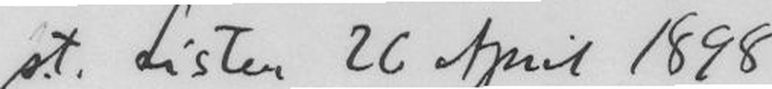st. Lister 26 April 1898
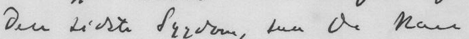den sidste Sygdom, saa De kan
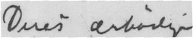Deres ærbødige
